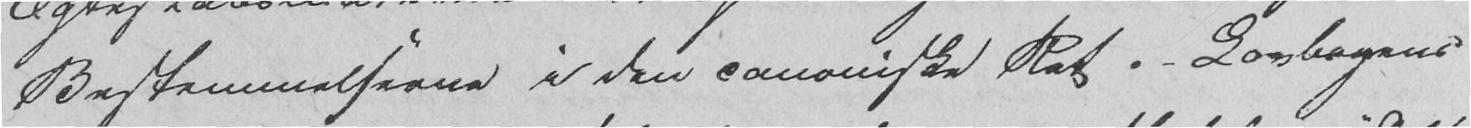Bestemmelserne i den canoniske Ret. - Lovbogens
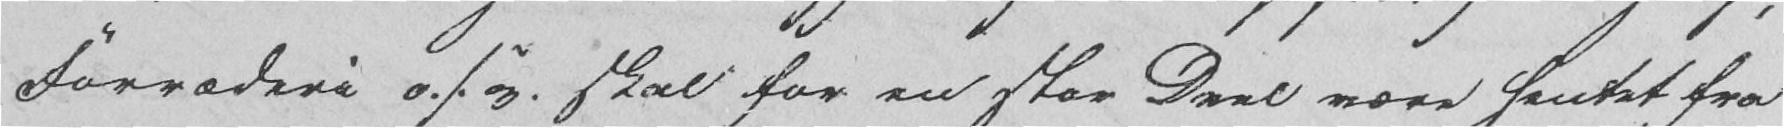Forræderi o. s. v. skal for en stor Deel være hentet fra
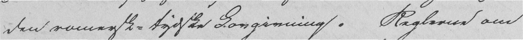den romersk-tydske Lovgivning. Reglerne om
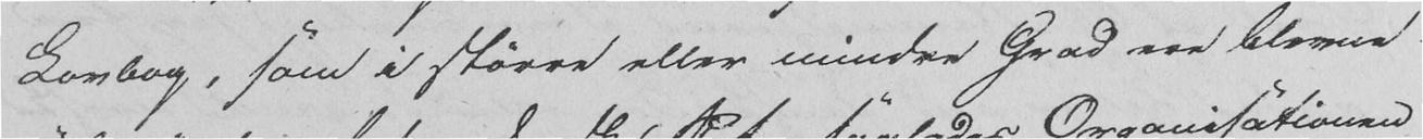Lovbog, som i større eller mindre Grad ere blevne
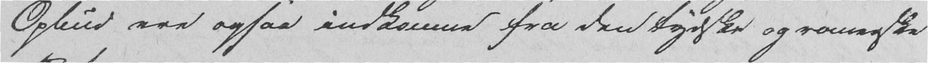Opbud ere ogsaa indkomne fra den tydske og romerske
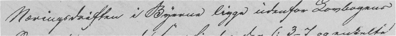Næringsdriften i Byerne ligge udenfor Lovbogens
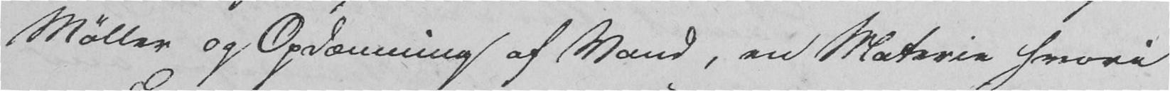Møller og Opdæmning af Vand, en Materie hvori
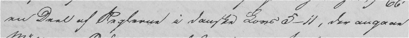en Deel af Reglerne i danske Lovs 5-11, der angaae
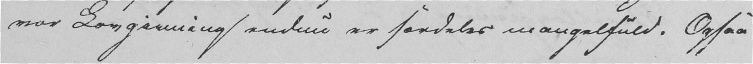vor Lovgivning endnu er særdeles mangelfuld. Ogsaa
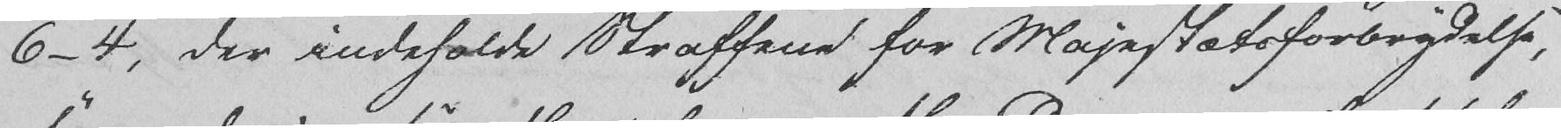6-4, der indeholde Straffene for Majestætsforbrydelse,
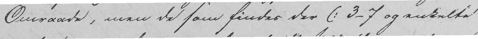Omraade, men de som findes der (: 3-7 og enkelte
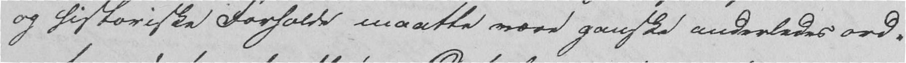og historiske Forholde maatte være ganske anderledes ord-
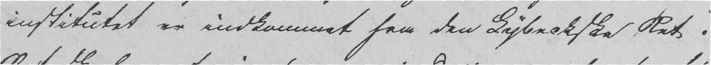institutet er indkommet fra den Lybeckske Ret.
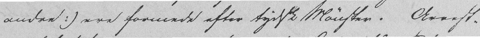andre :) ere formede efter tydsk Mønster. Arrest-
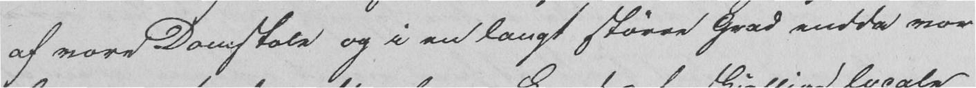af vore Domstole og i en langt større Grad endda vor
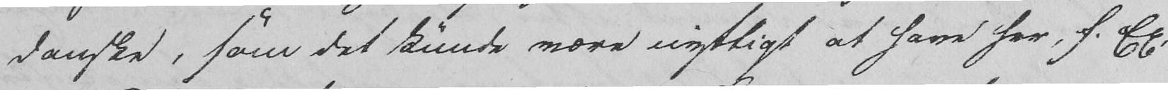danske, som det kunde være nyttigt at have her, f. Ex.
